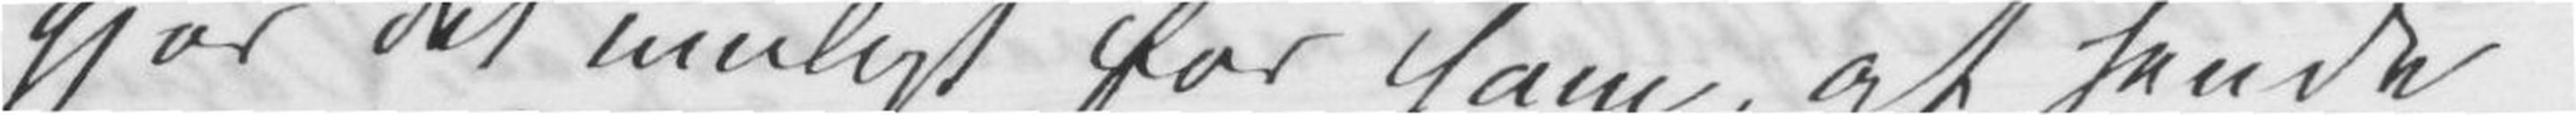gjør det muligt for ham, at sende
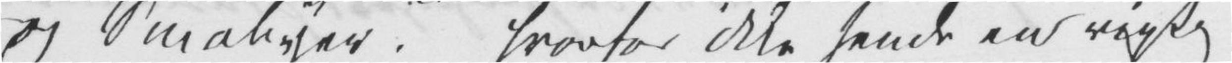og Småbyer? Hvorfor ikke sende en rigtig
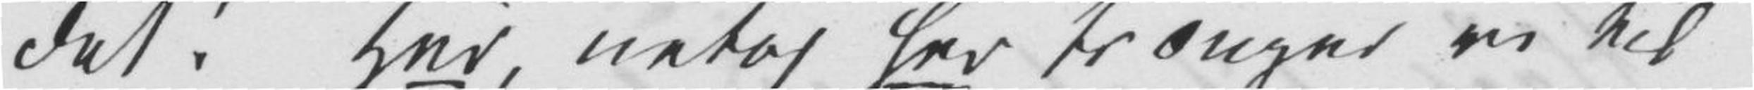det! Her, netop her trænger vi til
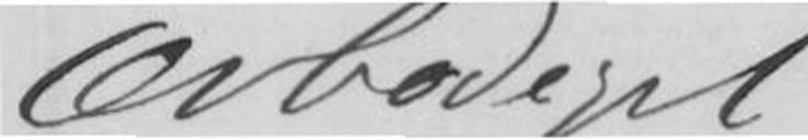Ærbødigst
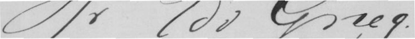Hr Edv Grieg.
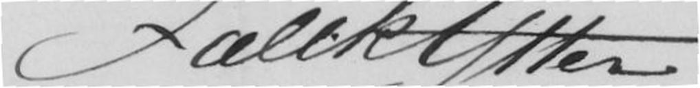Fallk Ytter.
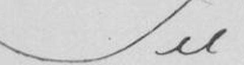Til
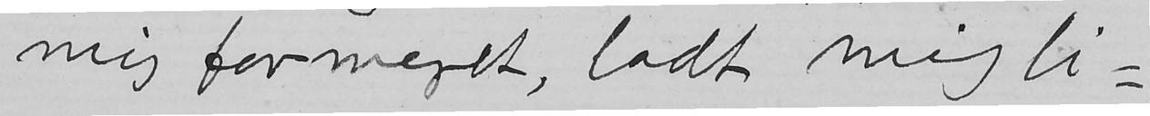mig formeret, ladt mig li-
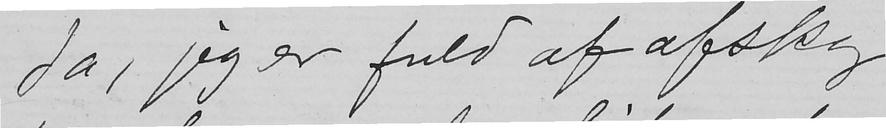ja, jeg er fuld af afsky
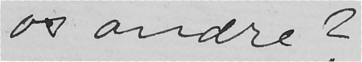os andre?
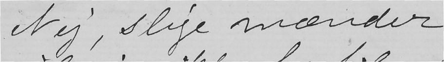Nej, slige mænder
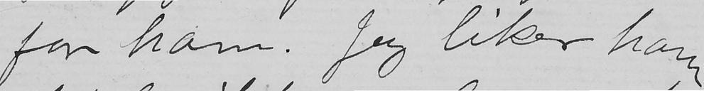for ham. Jeg liker ham
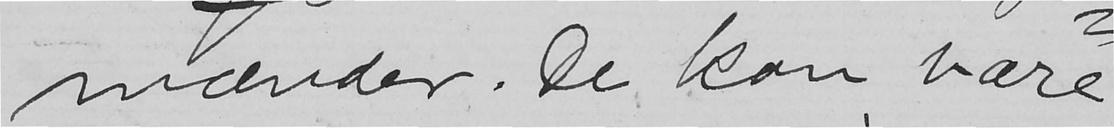mænder. De kan være
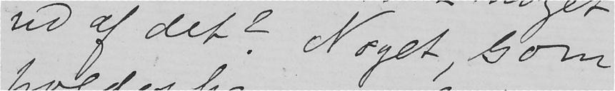ud af det? Noget, som
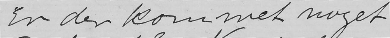Er der kommet noget
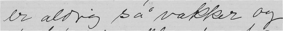er aldrig så vakker og
